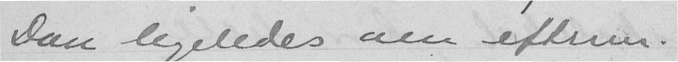Don ligeledes om efterm.
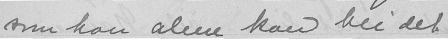som han alene kan bli det
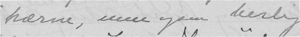trærne, men ogsaa herlig
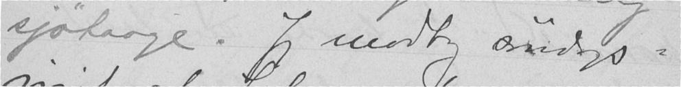sjøtaage. Jeg modtog søndags-
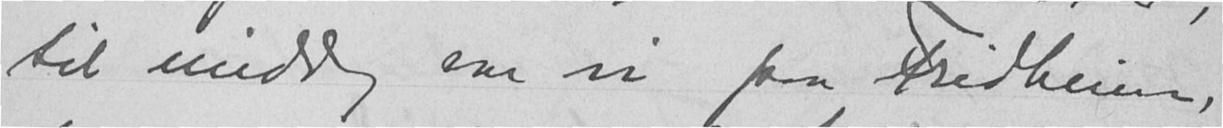til middag var vi paa Fridheim,
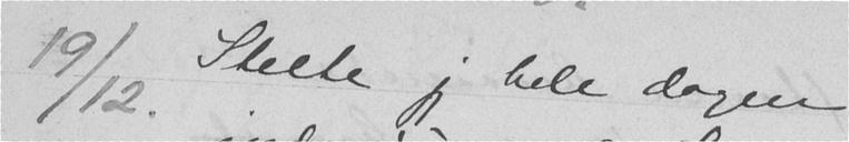19/12 Stelte jeg hele dagen
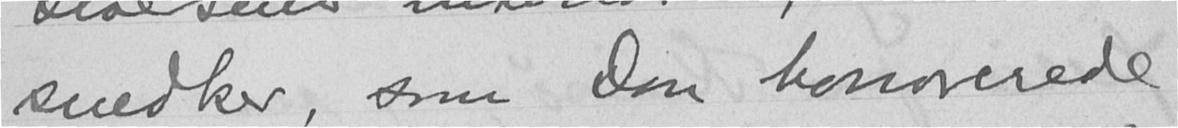snedker, som Don honorerede
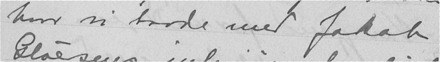hvor vi havde med Jakob
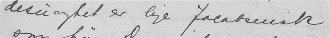desuagtet er lige Jacobsensk
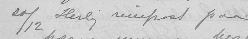20/12 Herlig rimfrost paa
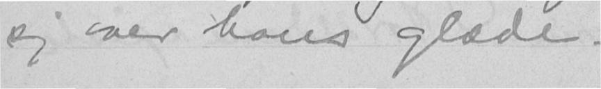sig over hans glæde.
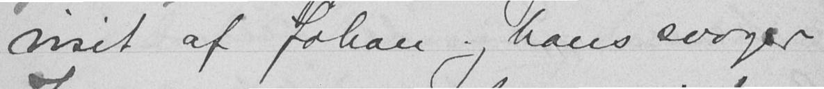visit af Johan og hans svoger
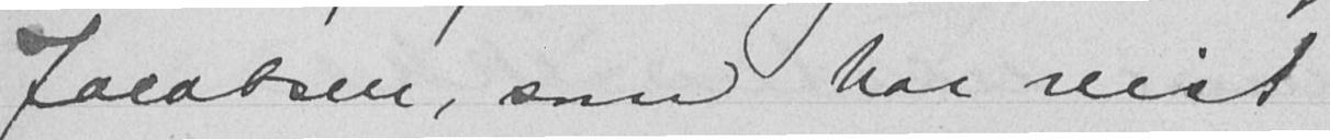Jacobsen, som har reist
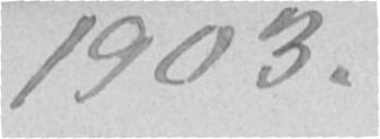1903.
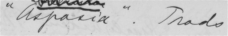"Aspasia". Trods
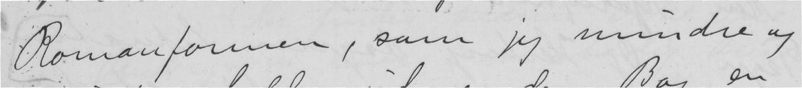Romanformen, som jeg mindre og
